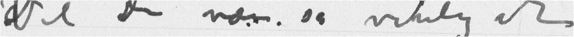Vil De være så venlig at
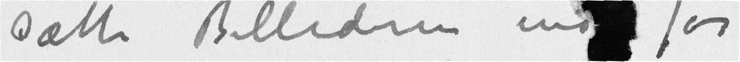sætte Billederne for
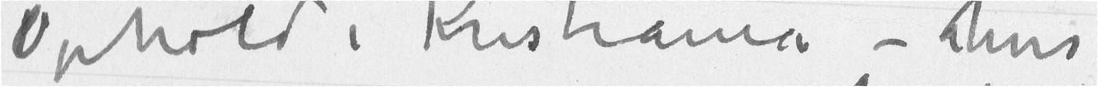Ophold i Kristiania – hvis
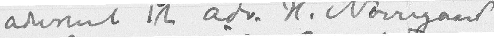adresseret til Adv. H. Nørregaard
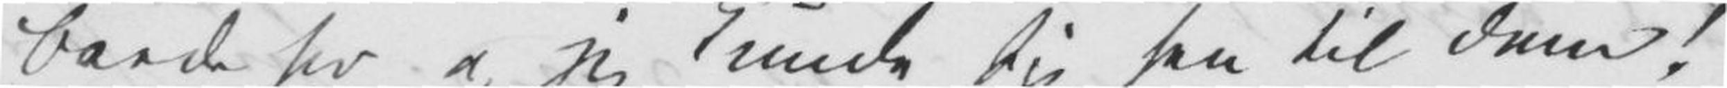boede her og jeg kunde ty hen til Dem!
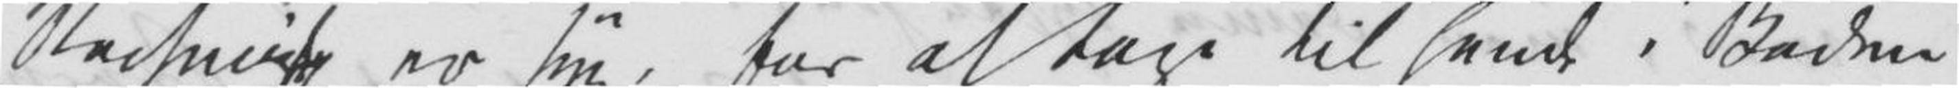Rechnitz er syg, for at tage til hende i Baden
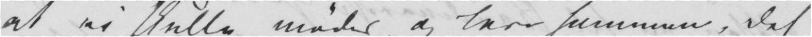at vi skulle mødes og leve sammen. Det
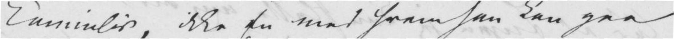Kaminliv, ikke En med hvem han kan gaa
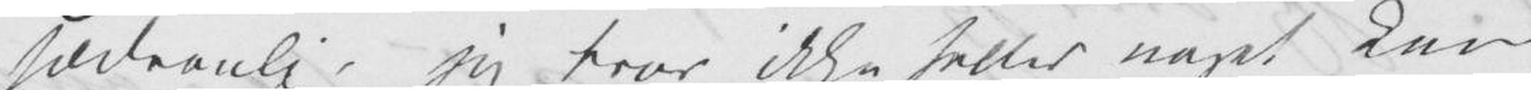sædvanlig, jeg tror ikke heller noget kan
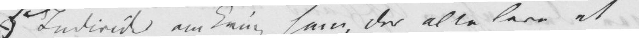5 Individ omkring ham, der alle leve et
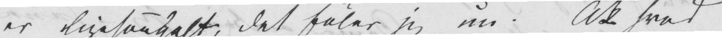er ligesaagalt, det føler jeg nu. Ak hvad
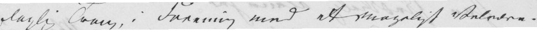daglig Tvang, i Forening med et mageligt Velvære.
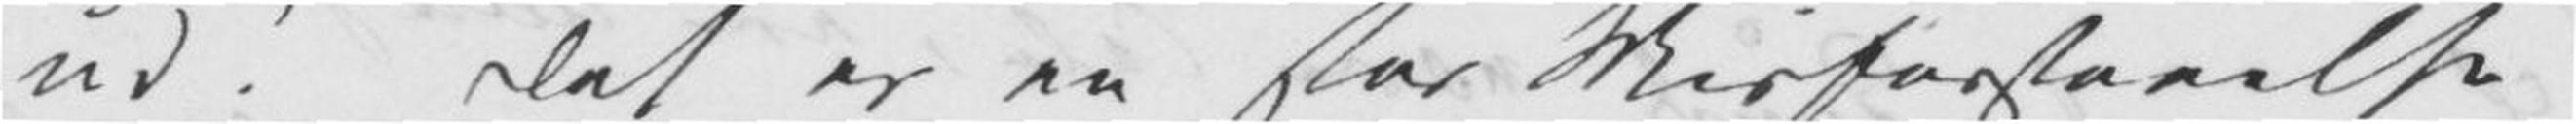ud! Det er en stor Misforstaaelse
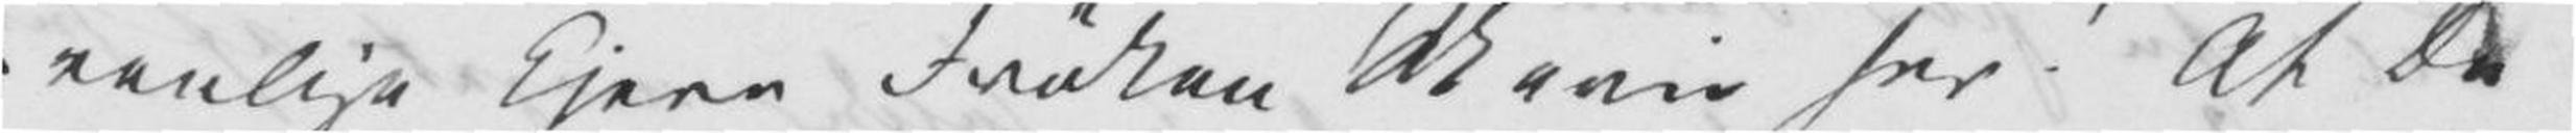venlige kjere Frøken Marie her! At De
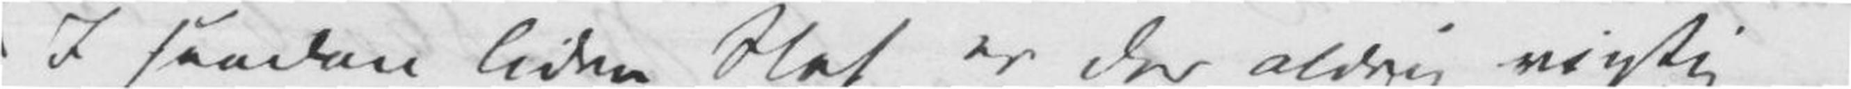(I saadan liden Stat er der aldrig rigtig
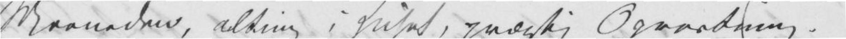Maaneden, alting i Huset, prægtig Opvartning.
